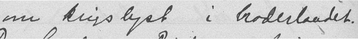om krigslyst i broderlandet.
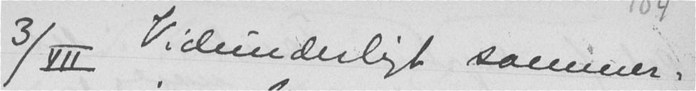3/VII Vidunderligt sommer-
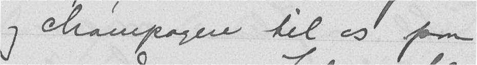og champagne til os paa
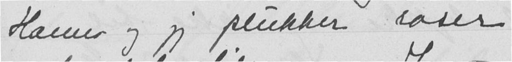Hanna og jeg plukker roser
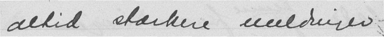altid stærkere meldinger
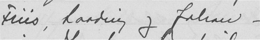Friis, Laading og Johan -
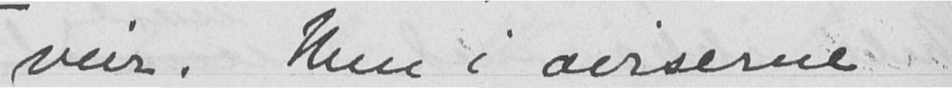veir. Men i aviserne
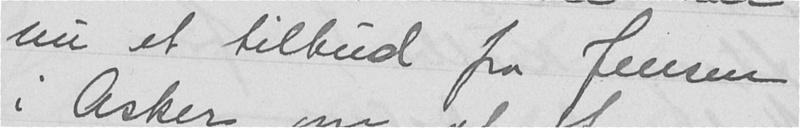nu et tilbud fra Jensen
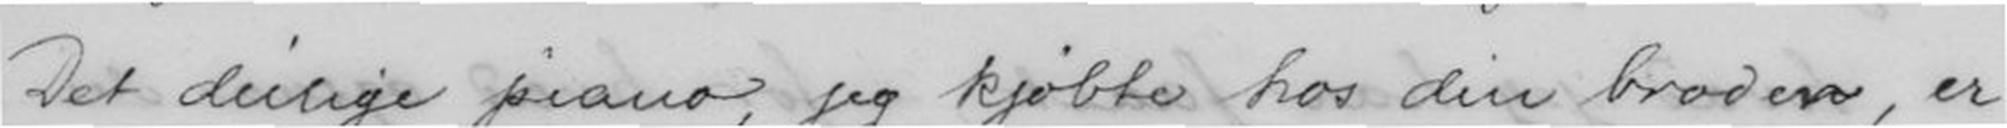Det deilige piano, jeg kjøbte hos din broder, er
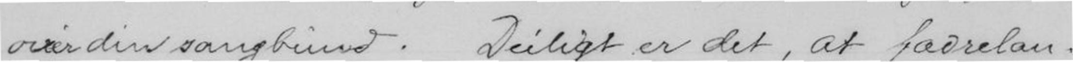over din sangbund. Deiligt er det, at fædrelan-
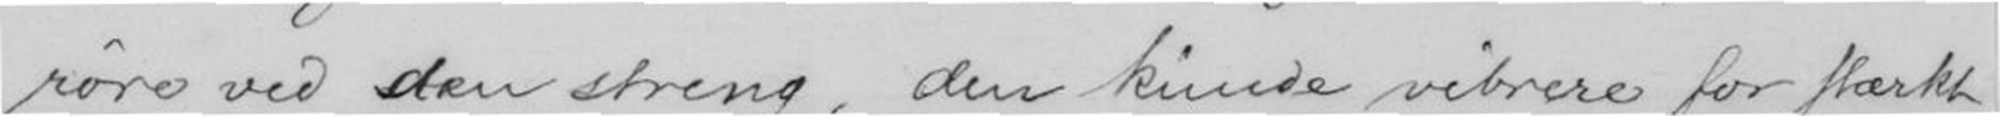røre ved den streng, den kunde vibrere for stærkt
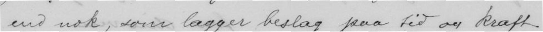end nok, som lægger beslag paa tid og kraft.
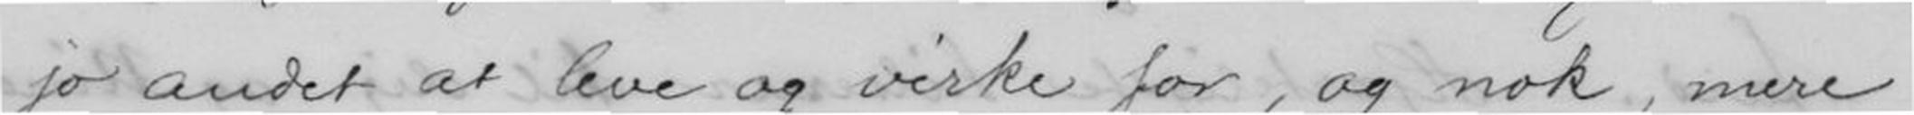jo andet at leve og virke for, og nok, mere
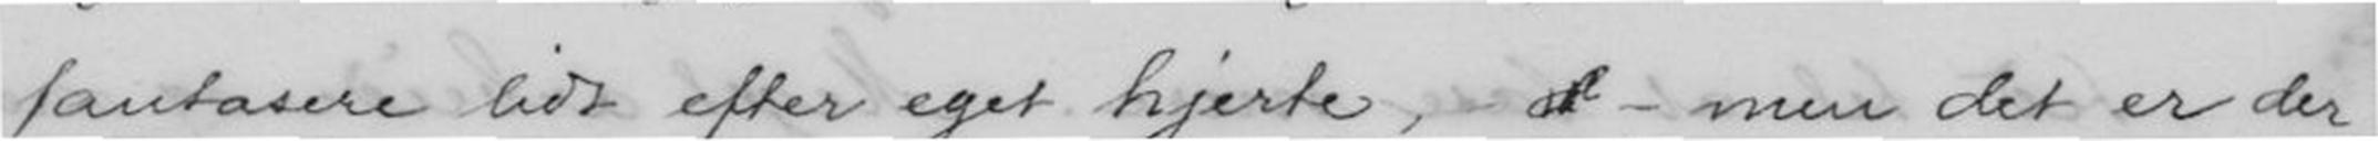fantasere lidt efter eget hjerte, - men det er der
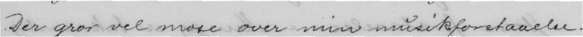Der gror vel mose over min musikforstaaelse.
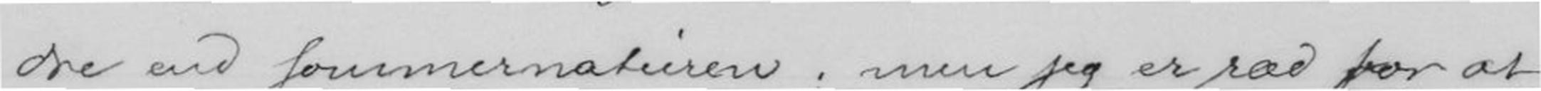dre end sommernaturen; men jeg er ræd for at
